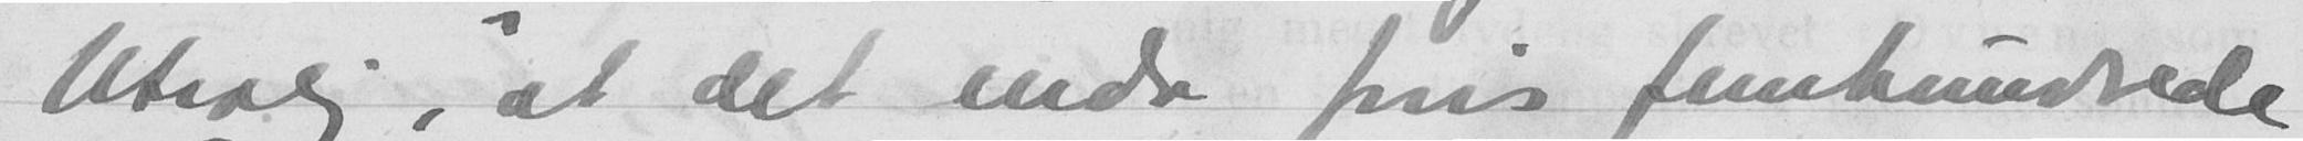Utrolig, at det enda fins femhundrede
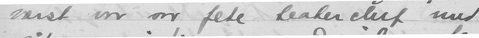værst var vor fete teaterchef med
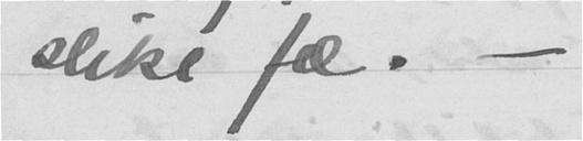slike fæ. -
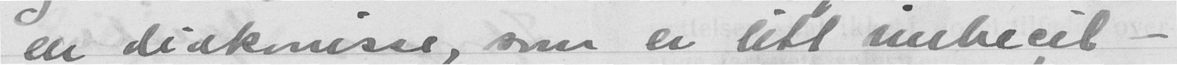en diakonisse, som er litt imbecil -
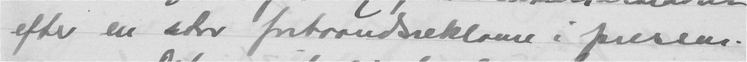efter en stor forhaandsreklame i pressen.
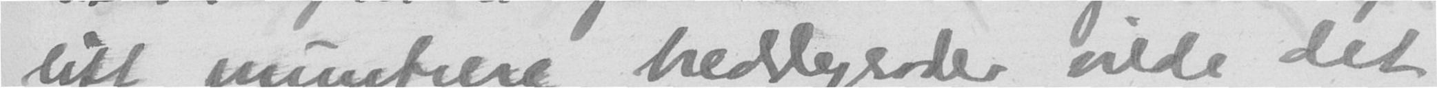litt muntrere breddegrader vilde det
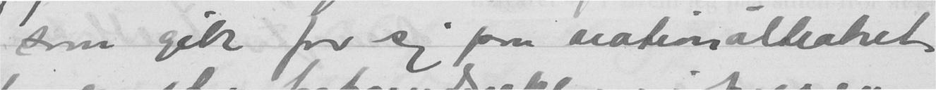som gik for sig paa nationaltheatret
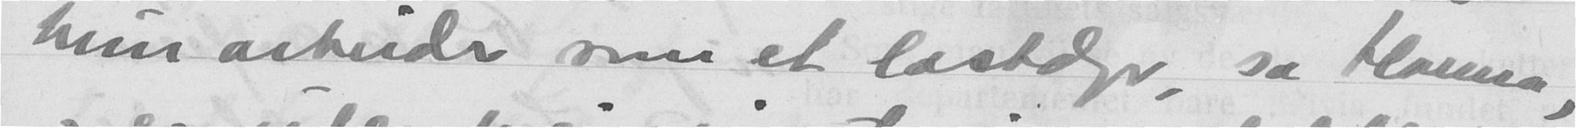hun arbeider som et lastedyr, sa Hanna,
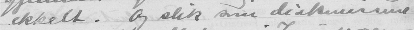ekkelt. Og slik som diakonissene
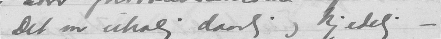Det var utrolig daarlig og kjedelig -
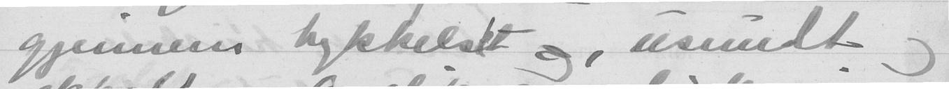gjennem hykkelskt og, usundt og
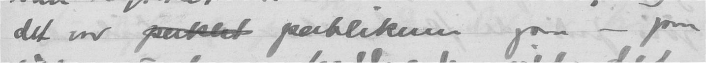det var pubsent publikum ogsaa - paa
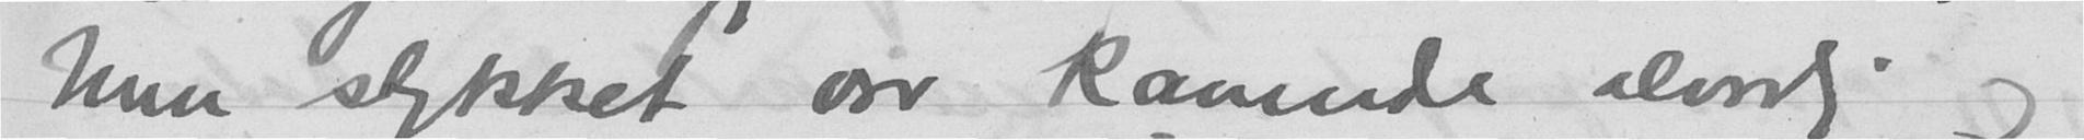Men stykket var kavende alvorlig og
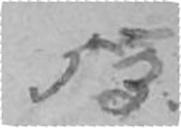53.
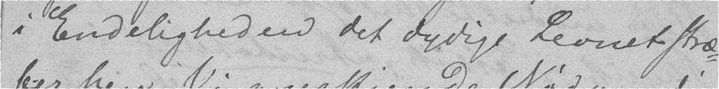i Endeligheden det dydige Levnet stræ-
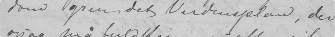dom grundet Verdensplan, der
